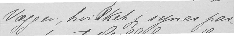Væggen, hvilket jeg synes pas-
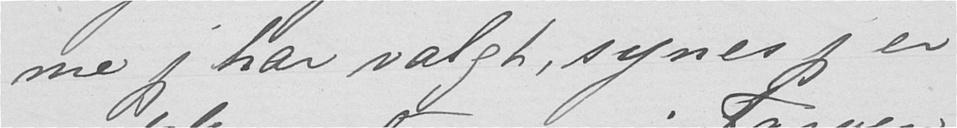me jeg har valgt, synes jeg er
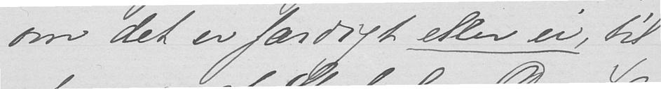om det er færdigt eller ei, til
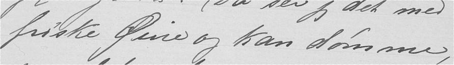friske Øine og kan dømme,
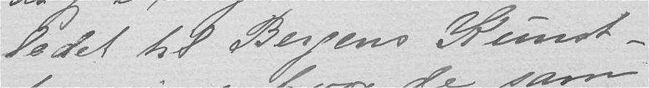ledet til Bergens Kunst-
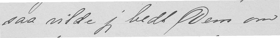saa vilde jeg bedt Dem om
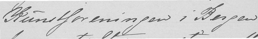Kunstforeningen i Bergen
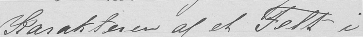Karakteren af et Felt i
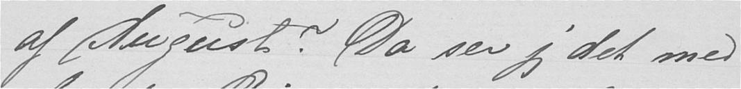af August? Da ser jeg det med
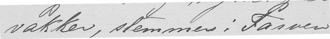vakker, stemmer i Farven
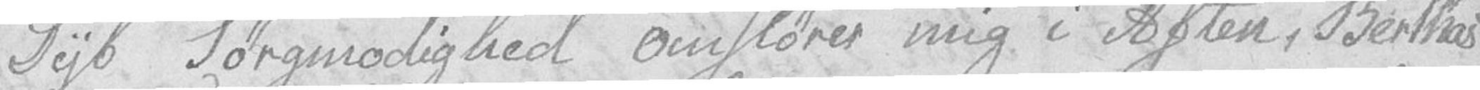Dyb Sørgmodighed omslører mig i Aften, Berthas
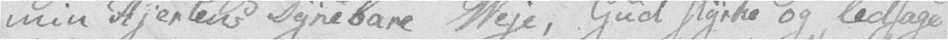min Hjertens Dyrebare Veje, Gud styrke og ledsage
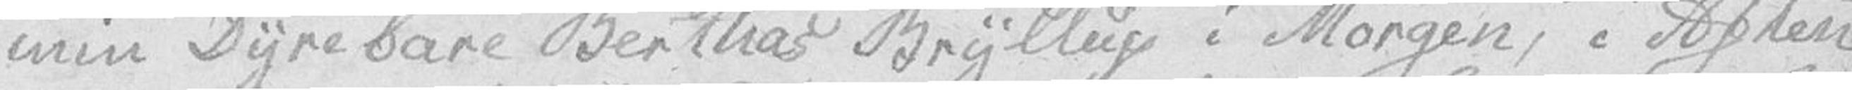min Dyrebare Berthas Bryllup i Morgen, i Aften
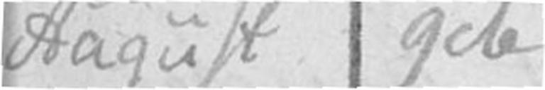August 9de
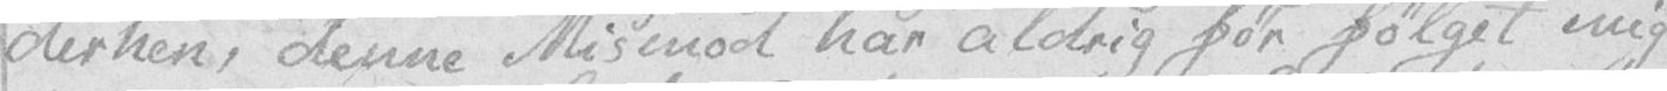derhen, denne Mismod har aldrig før følget mig
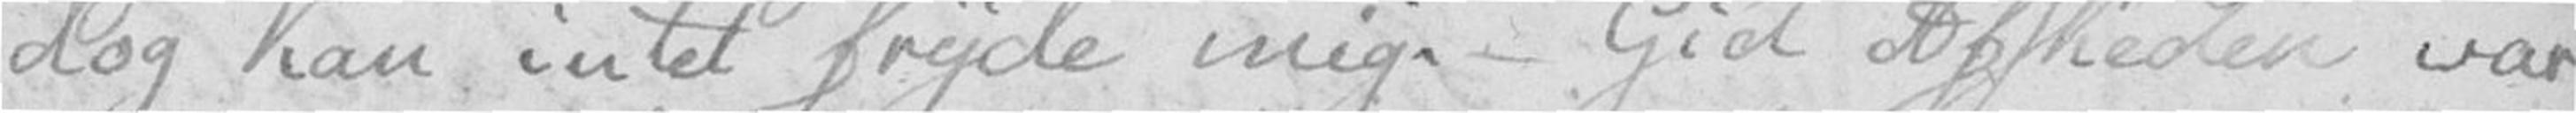dog kan intet fryde mig. – Gid Afskeden var
